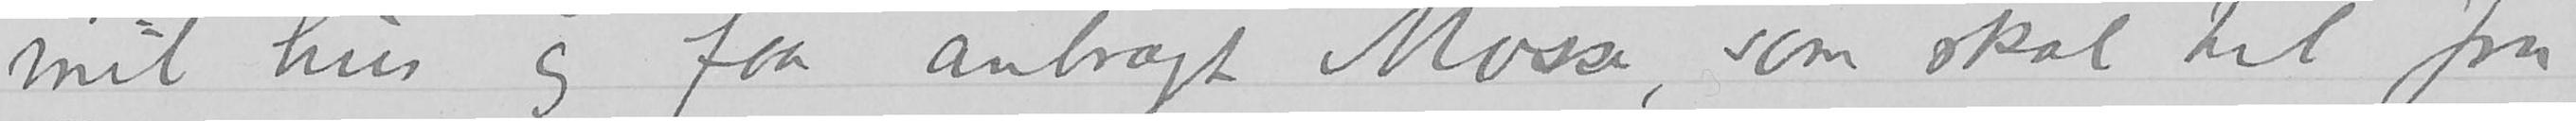mit hus og faa anbragt Mosse, som skal til fru
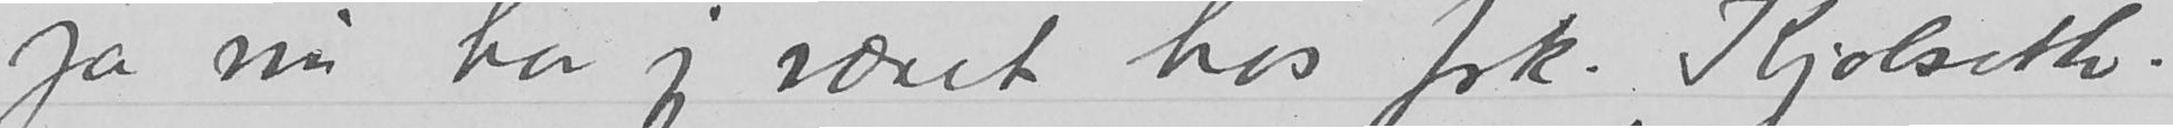ja nu har jeg været hos frk. Kjølseth.
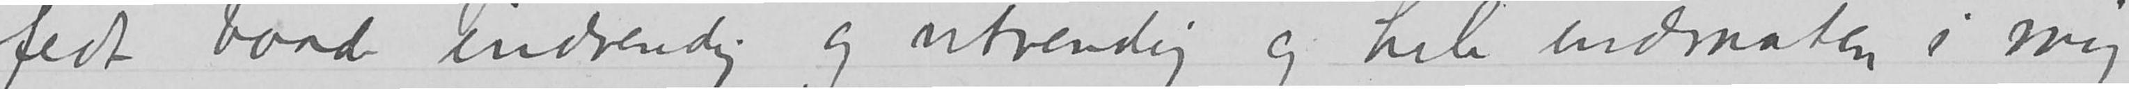fedt baade indvendig og utvendig og hele indmaten i mig
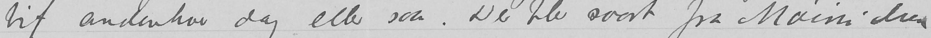bif andenhver dag eller saa. Der blev svart fra Møinichen
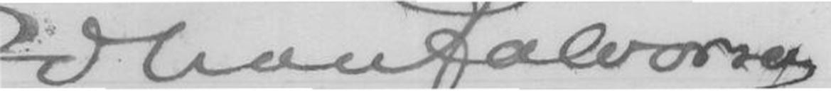Johan Halvorsen
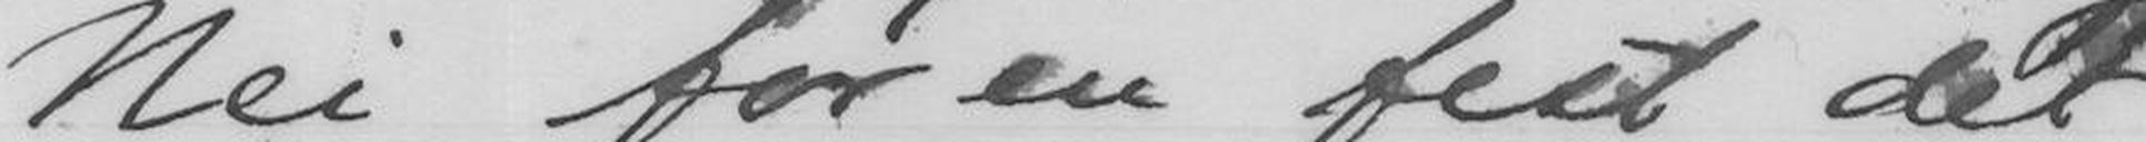Nei for en fest det
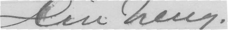Din heng.
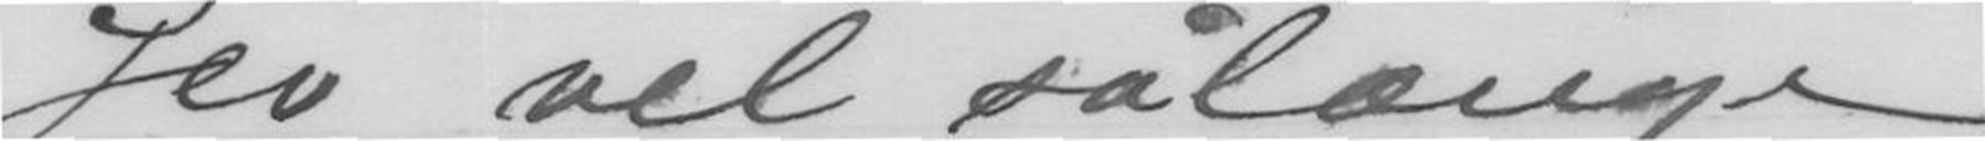Lev vel sålænge
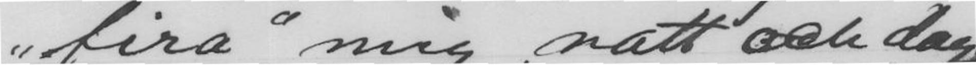fira mig natt och dag
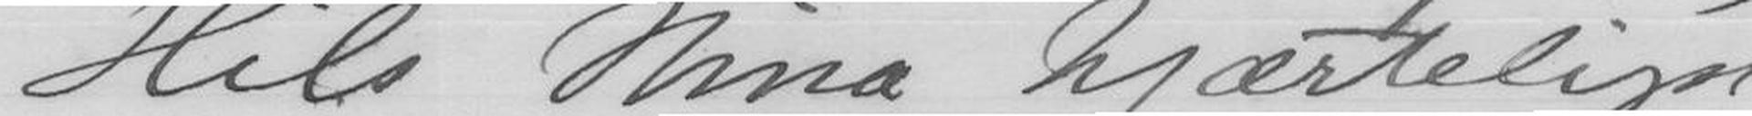Hils Nina hjærteligst
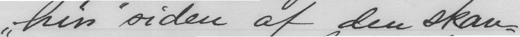hin siden af den skan-
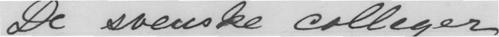De svenske colleger
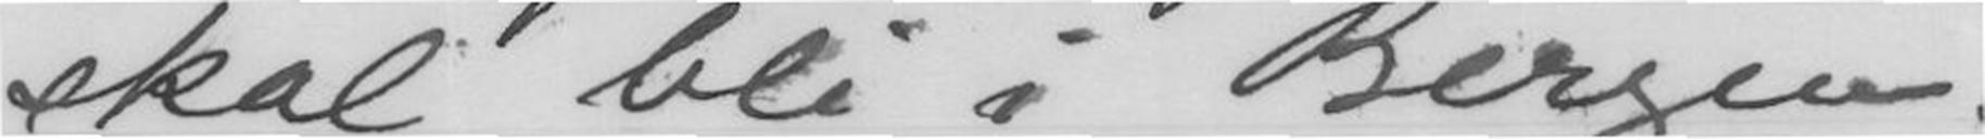skal bli i Bergen.
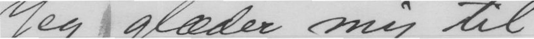Jeg glæder mig til
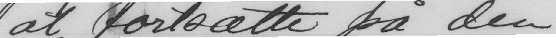at fortsætte på den
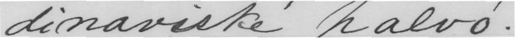dinaviske halvø.
